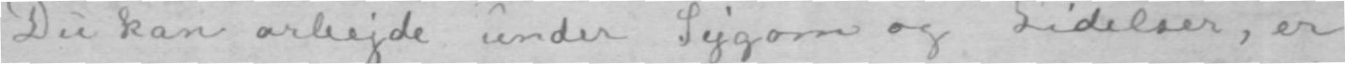Du kan arbejde under Sygom og Lidelser, er
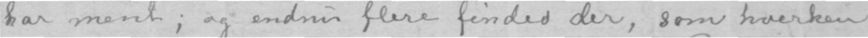har ment; og endnu flere findes der, som hverken
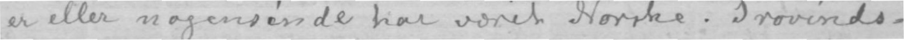er eller nogensinde har været Norske. Provinds-
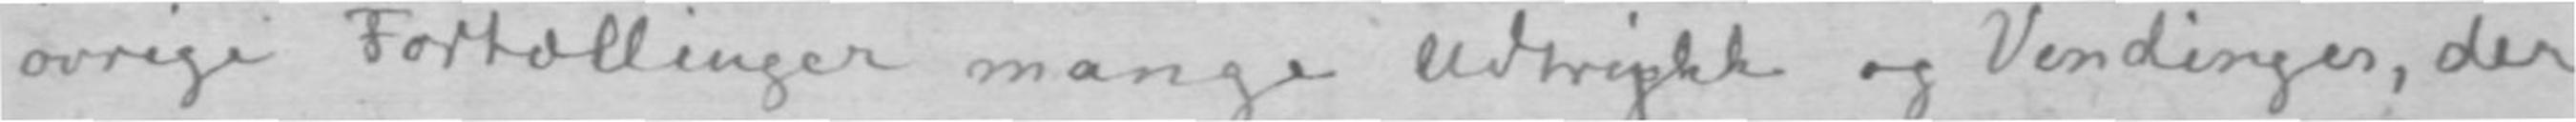øvrige Fortællinger mange Udtrykk og Vendinger, der
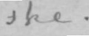ske.
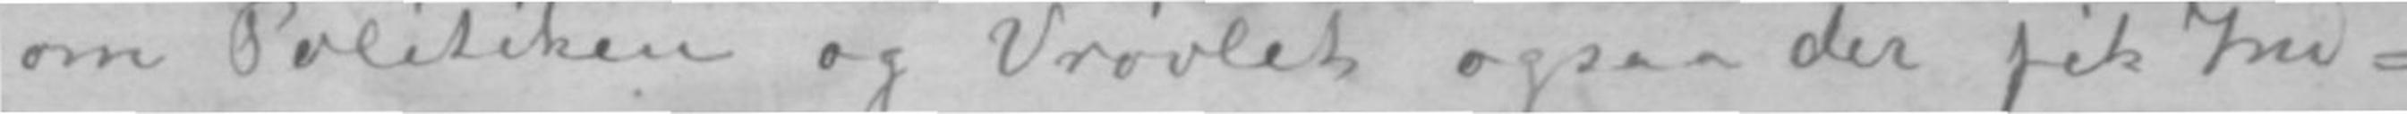om Politiken og Vrøvlet ogsaa der fik Ind-
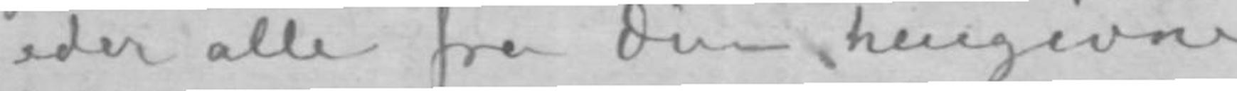eder alle fra Din hengivne
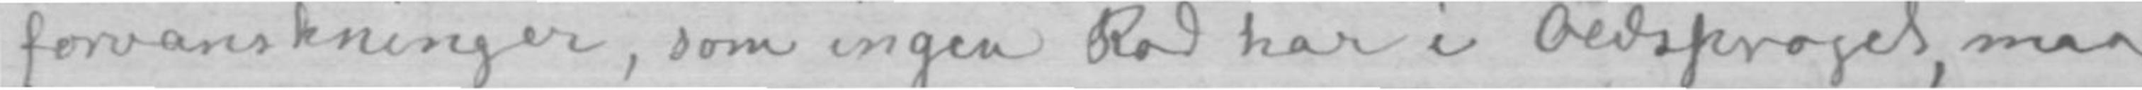forvanskninger, som ingen Rod har i Oldsproget, maa
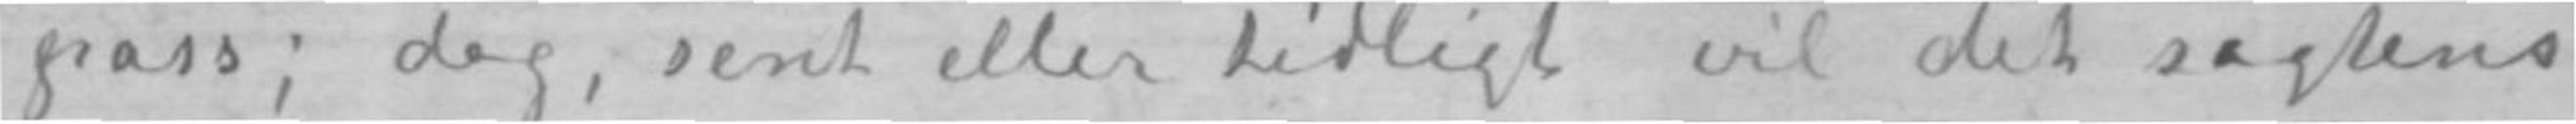pass; dog, sent eller tidligt vil det sagtens
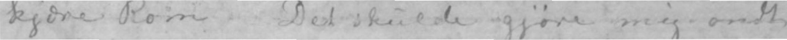kjære Rom Det skulde gjøre mig ondt
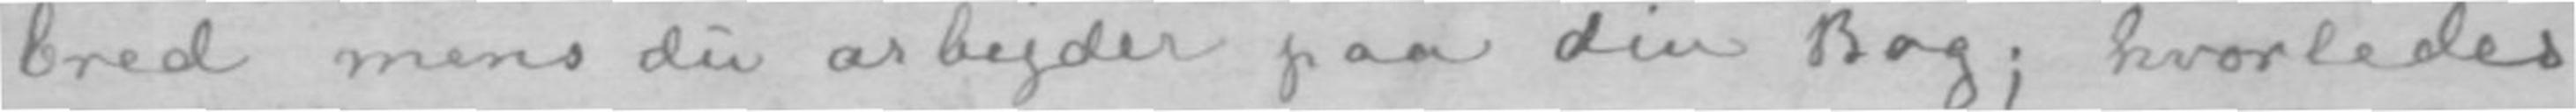bred mens du arbejder paa din Bog; hvorledes
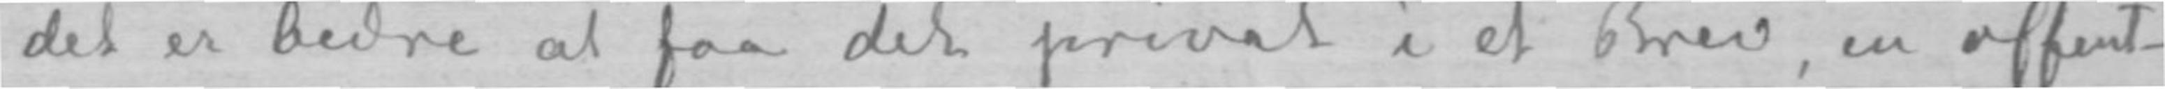det er bedre at faa det privat i et Brev, en offent-
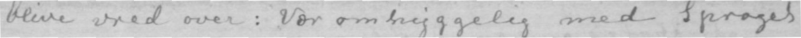blive vred over: Vær omhyggelig med Sproget
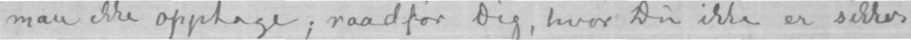man ikke opptage; raadfør Dig, hvor Du ikke er sikker,
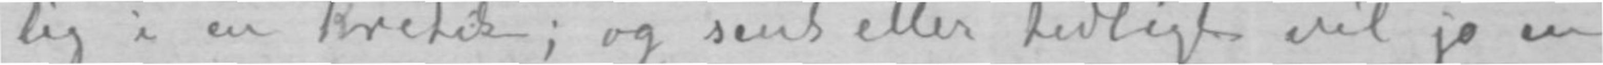lig i en Kritik; og sent eller tidligt vil jo en
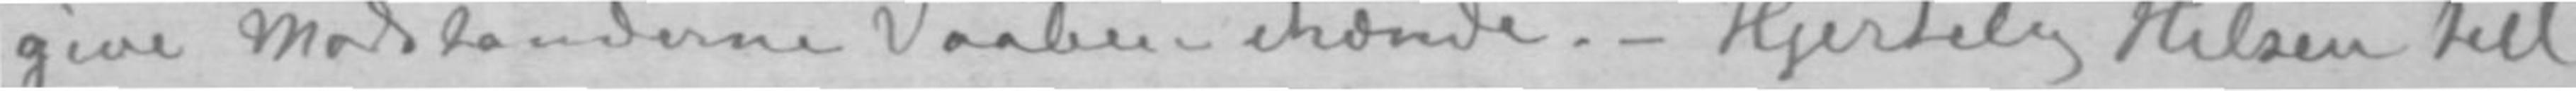give Modstanderne Vaaben ihænde.- Hjertelig Hilsen till
